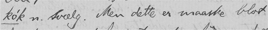kók n. svælg. Men dette er maaske blot
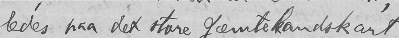ledes paa det store Jæmtelandskart
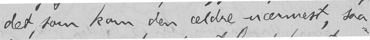det, som kom den ældre nærmest, saa-
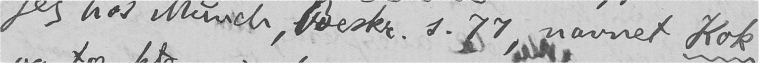jeg hos Munch, Beskr. s. 77, navnet Kok
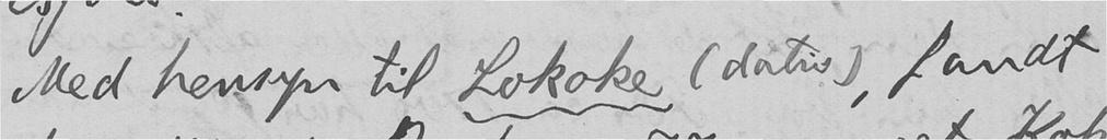Med hensyn til Lokoke (dativ), fandt
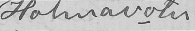Holmavatn.
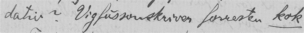dativ? Vigfusson skriver forresten kok.
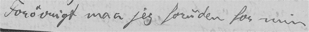Forøvrigt maa jeg foruden for min
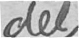del
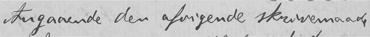Angaaende den afvigende skrivemaade
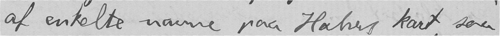af enkelte navne paa Holms kart, saa
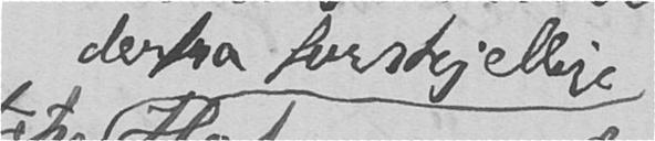derfra forskjellige
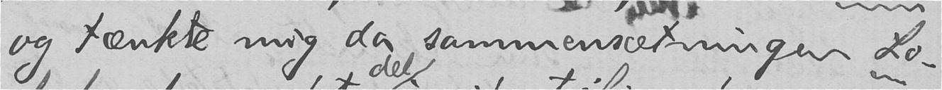og tænkte mig da sammensætningen Lo-
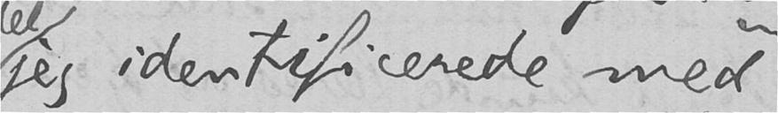jeg identificerede med
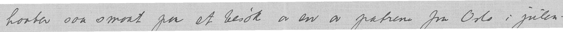haaber saa smaat paa et besøk av en av patrene fra Oslo i julen.
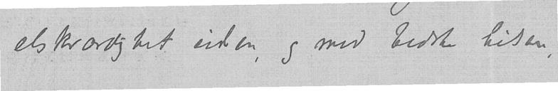elskværdighet siden, og med bedste hilsen,
